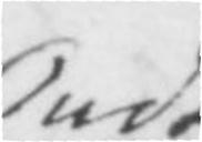Ondt
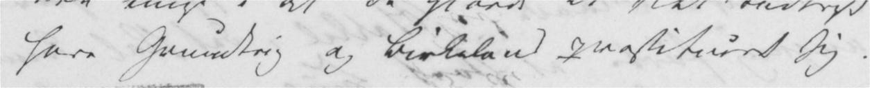have Grundtvig og Birkeland prostitueret sig.
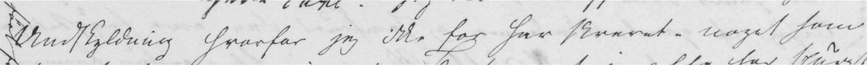Undskyldning hvorfor jeg ikke før har skrevet – noget som
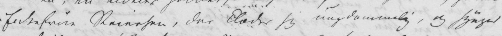Enkefrue Reiersen, der klæder sig ungdommelig, og synger
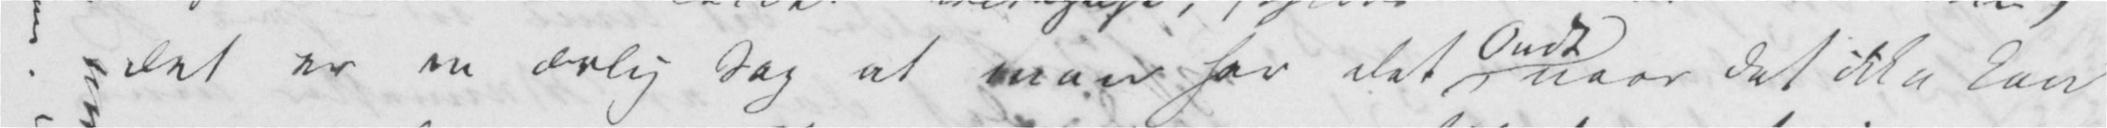det er en ærlig Sag at man har det , naar det ikke kan
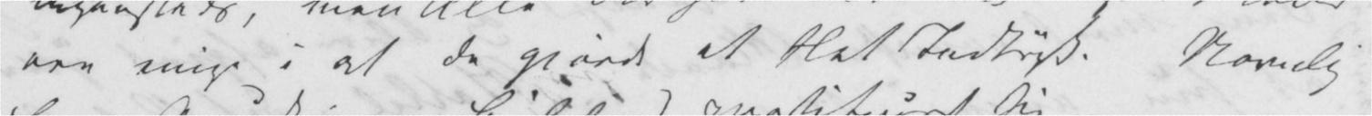ere enig i at de gjorde et slet Indtryk. Navnlig
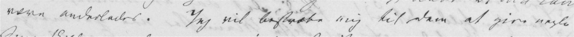være anderledes. Jeg vil bestræbe mig til Dem at give nogle
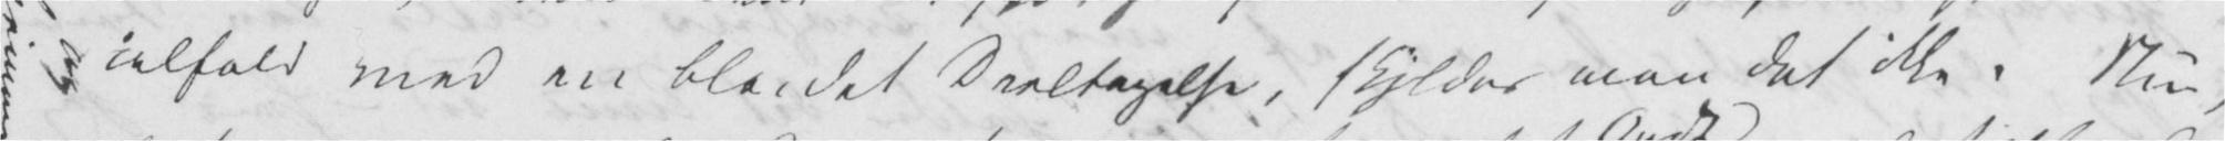ialfald med en blandet Deeltagelse, skylder man det ikke. Nu,
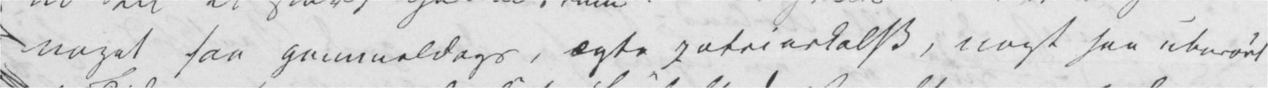noget saa gammeldags, ægte patriarkalsk, noget saa uberørt
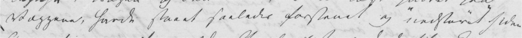Væggene, havde staaet saaledes forstenet og «nedstøvet» siden
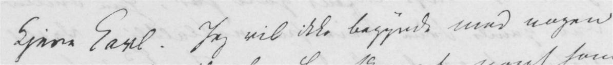Kjere Carl. Jeg vil ikke begynde med nogen
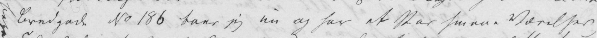Bredgade No 186 boer jeg nu og har et Par smaae Værelser
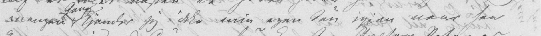mangen kjender jeg ikke min egen Søn igjen naar han
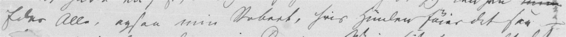Eder Alle, ogsaa min Robert, hvis Himlen føler det saa –
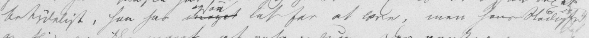betydeligt, han har meget let for at lære, men hans Stadighed
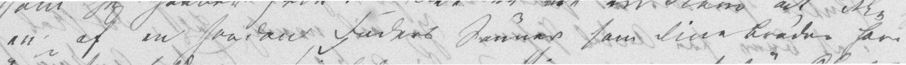en af en saadan Faders Sønner som Dine Brødre have
